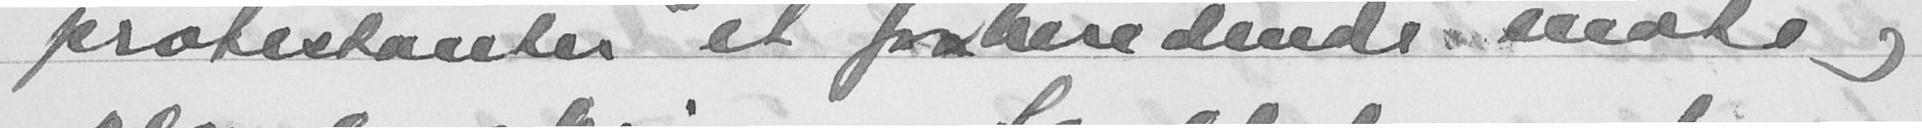protestanter et forberedende møte og
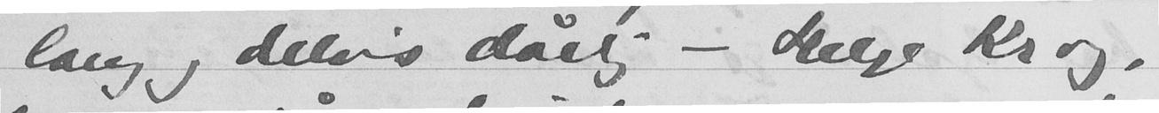lang, delvis dårlig - Helge Krog,
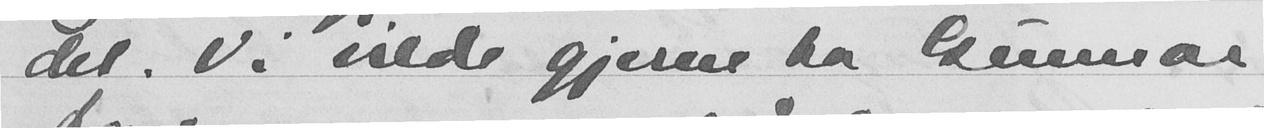det. Vi vilde gjerne ha Gunnar
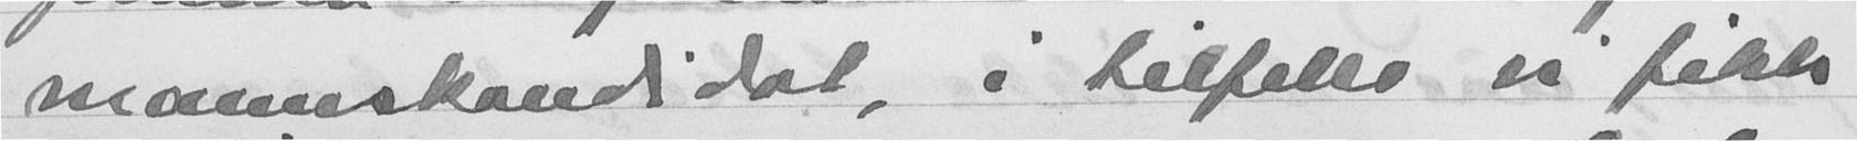mannskandidat i tilfelle vi fikk
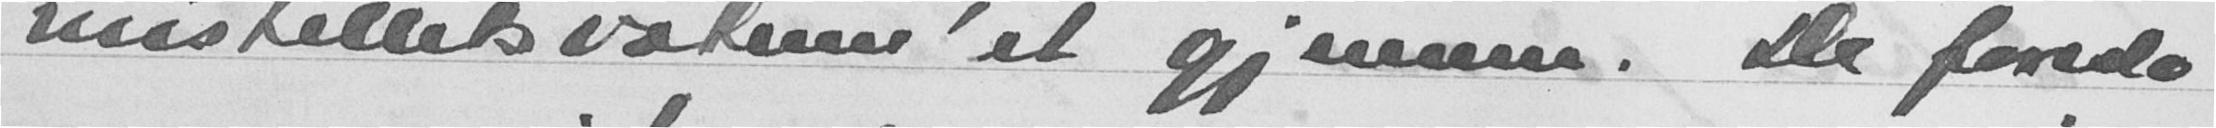mistillitsvotum'et gjennom. De foreslo
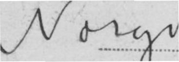Norge
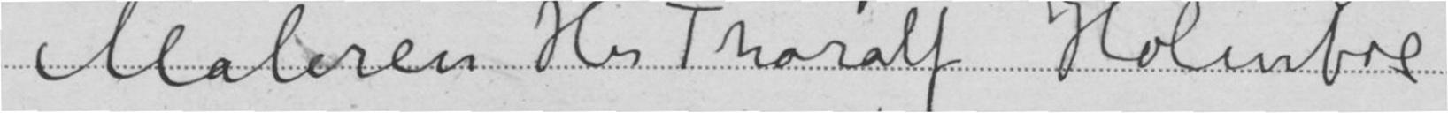Maleren Hr Thorolf Holmboe
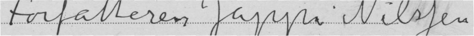Forfatteren Jappe Nilssen
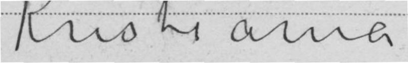Kristiania
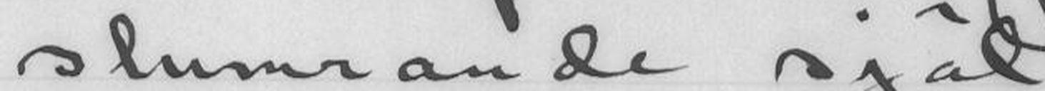slumrande själ
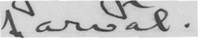farväl.
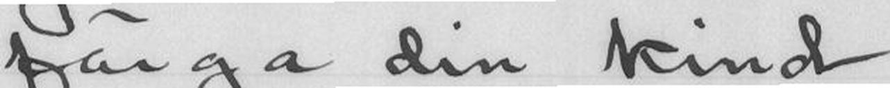färga din kind
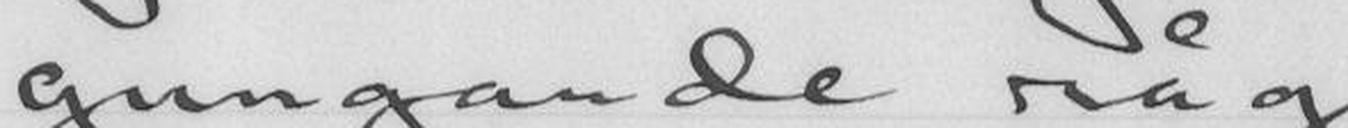gungande råg
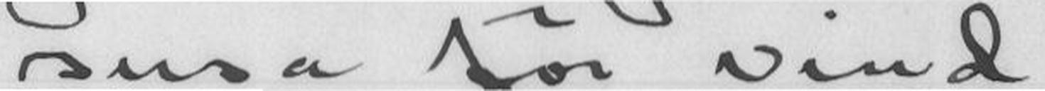susa för vind
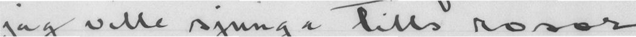jag ville sjunga tills rosor
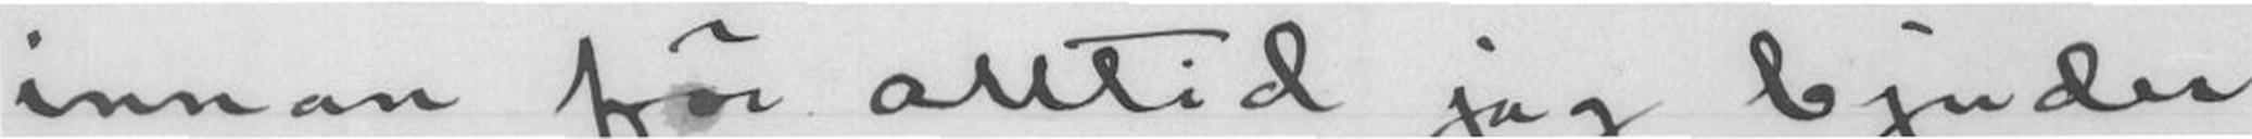innan för alltid jag bjuder
